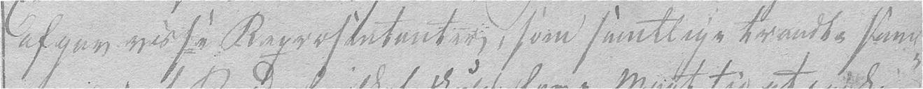afgav visse Repræsentanter, som samtlige traadte sam-
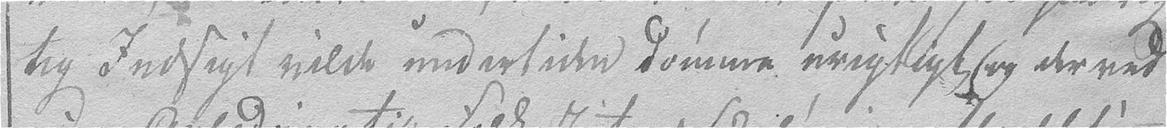tig Indsigt vilde undertiden dømme urigtigt og derved
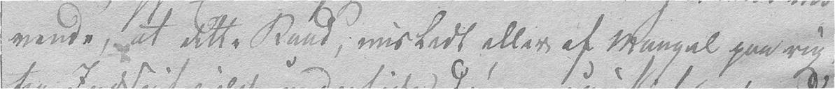vende, at dette Raad, misledt eller af Mangel paa rig-
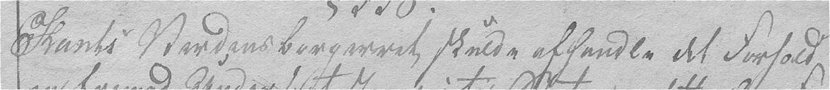Kants Verdensborgerret skulde afhandle det Forhold
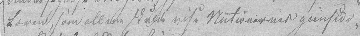Læren, som allene skulde vise Nationernes gjensidi-
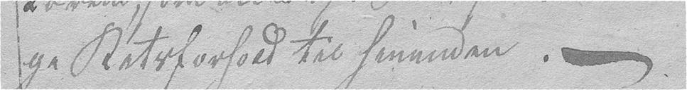ge Retsforhold til hinanden. –
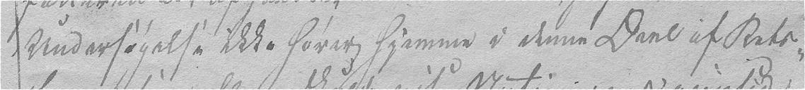Undersøgelse ikke hører hjemmer i denne Deel af Rets-
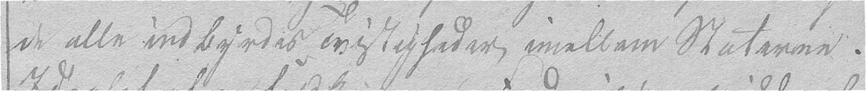de alle indbyrdes Tvistigheder imellem Staterne.
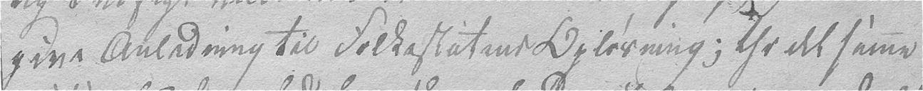give Anledning til Folkestatens Opløsning; thi det samme
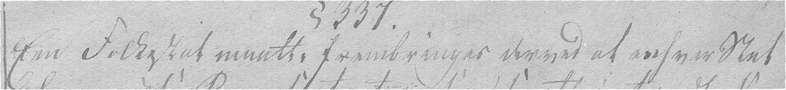En Folkestat maatte frembringes derved at enhver Stat
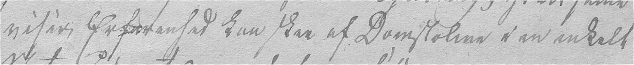viser Erfarenhed kan skee af Domstolene i en enkelt
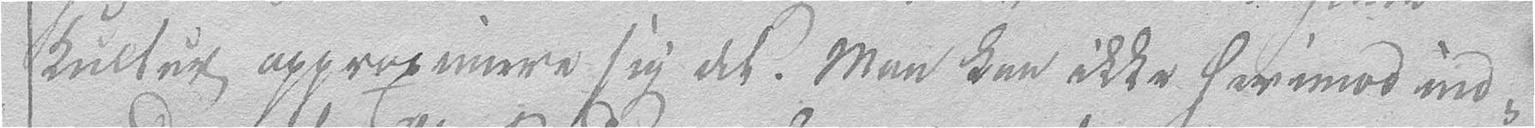Kultur approximere sig det. Man kan ikke herimod ind-
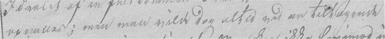opnaaes; men man vilde dog altid ved en tiltagende
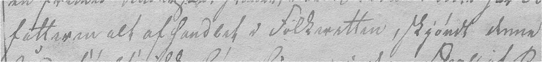fatteren alt afhandlet i Folkeretten, skjøndt denne
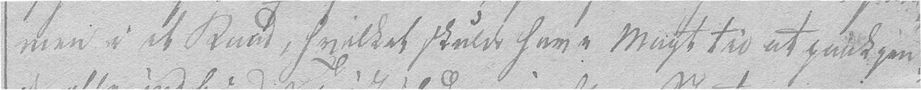men i et Raad, hvilket skulde have Magt til at paakjen-
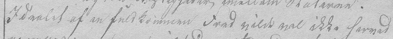Idealet af en fuldkommen Fred vilde vel ikke herved
